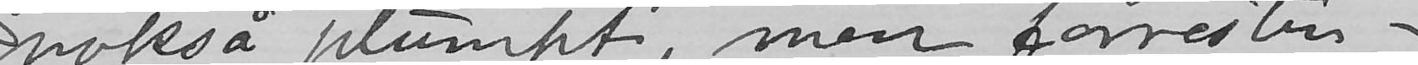nokså plumpt, men forresten-
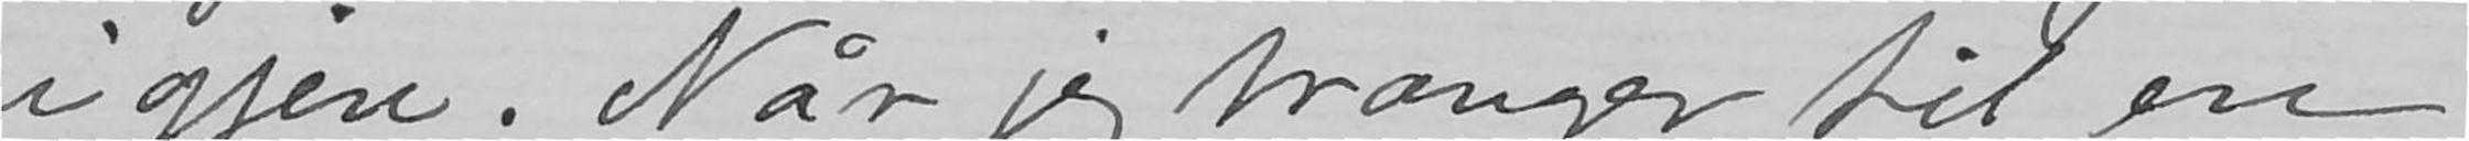igjen. Når jeg trænger til en
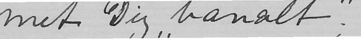met Dig "banalt".
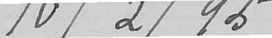10/2/95
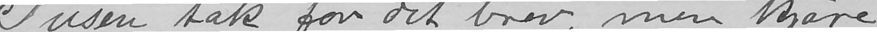Tusen tak for dit brev, min kjære
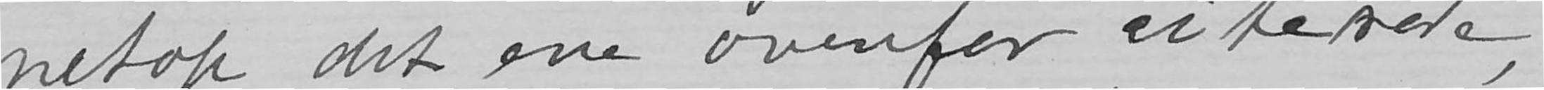netop det ene overfor citerede,
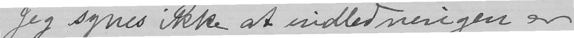Jeg synes ikke at indledningen er
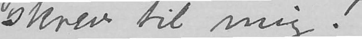skrev til mig!
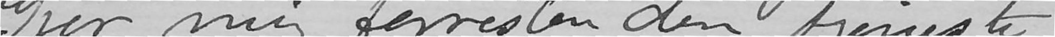Gjør meg forresten den tjeneste,
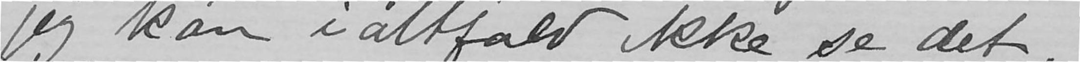jeg kan ialtfald ikke se det.
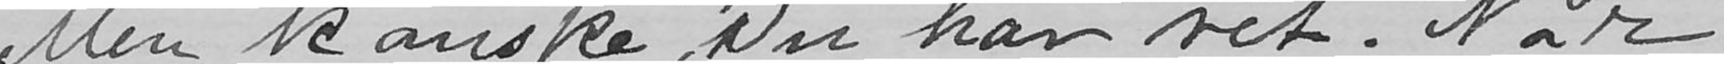Men kanske, Du har ret. Når
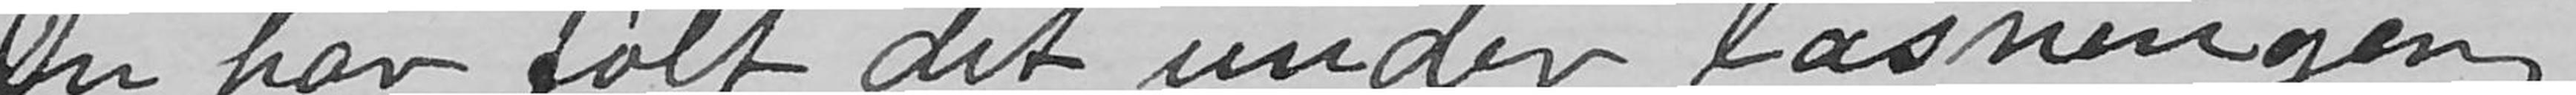Du har følt det under læsningen,
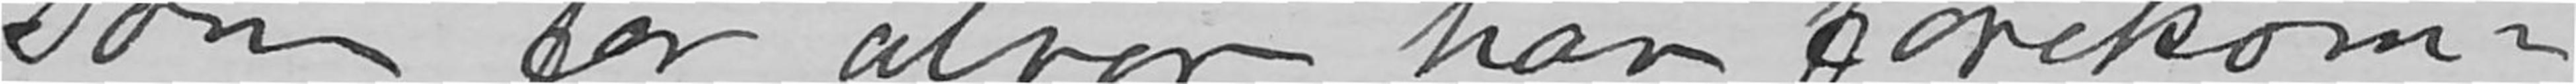som for alvor har forekom-
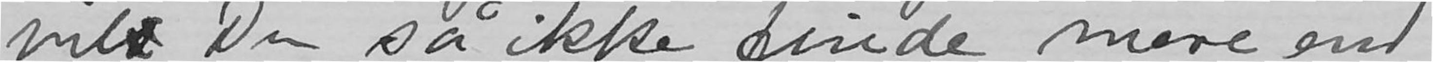vil Du så ikke finde mere end
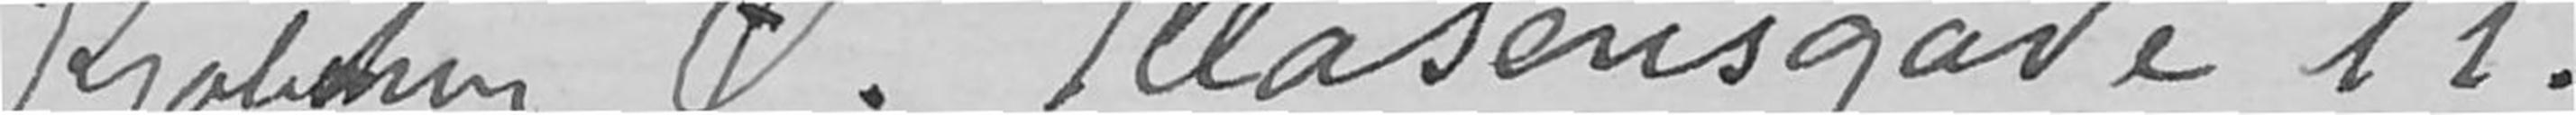Kjøbehvn Ø. Klasensgade 11.
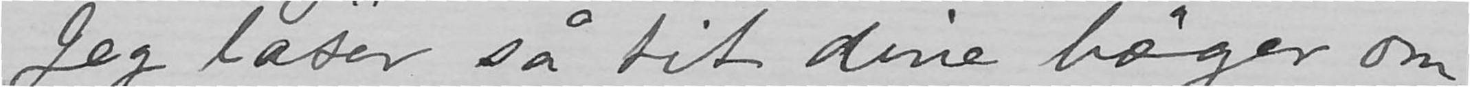Jeg læser så tit dine bøger om
